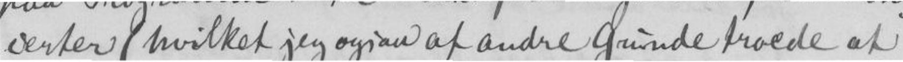certer (hvilket jeg ogsaa af andre Grunde troede at
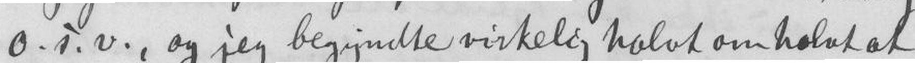o.s.v., og jeg begyndte virkelig halvt om halvt at
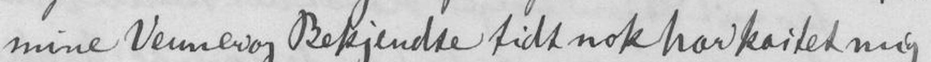mine Venner og Bekjendte tidt nok har kastet mig
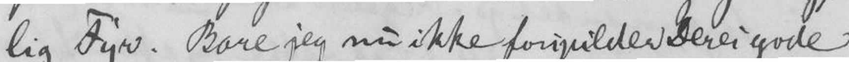lig Fyr. Bare jeg nu ikke forspillder Deres gode
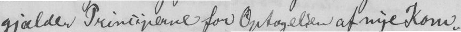gjælder Prinsiperne for Optagelsen af nye Kom-
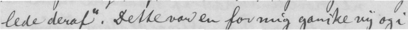lede deraf". Dette var en for mig ganske ny og i
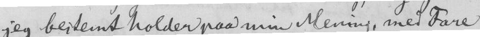jeg bestemt holder paa min Mening, med Fare
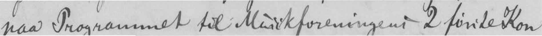paa Programmet til Musikforreningens 2 første Kon-
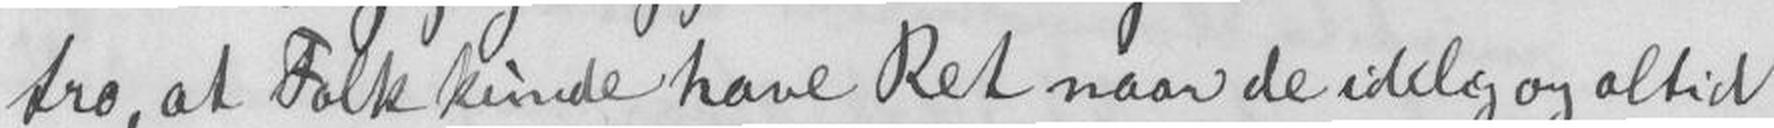tro, at Folk kunde have Ret naar de idelæg og altid
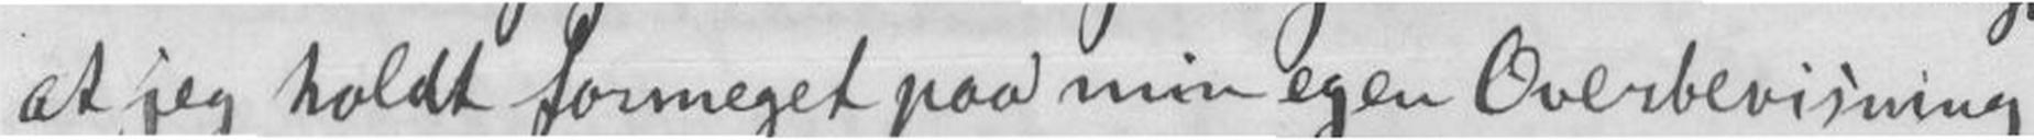at jeg holdt formeget paa min egen Overbevisning
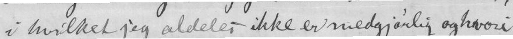i hvilket jeg aldeles ikke er medgjørlig og hvori
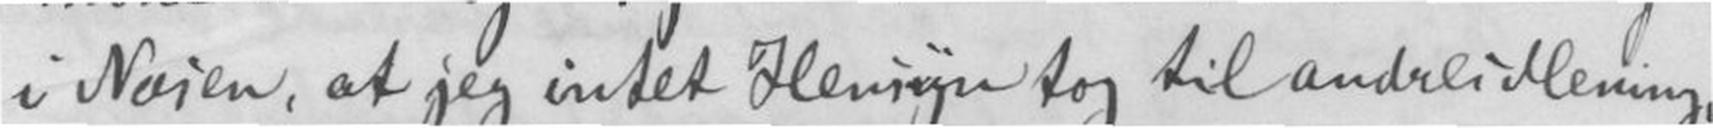i Nasen, at jeg intet Hensyn tog til andres Mening,
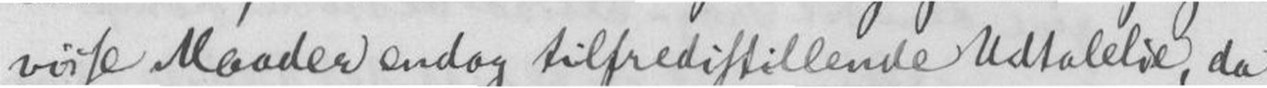visse Maader endog tilfredsdtillende Udtalelse, da
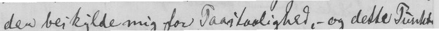der beskylder for Paastaalighed, - og dette Punkt
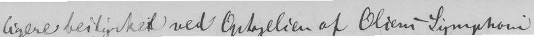legere bestyr alt ved Optagelsen af Olsens Symphoni
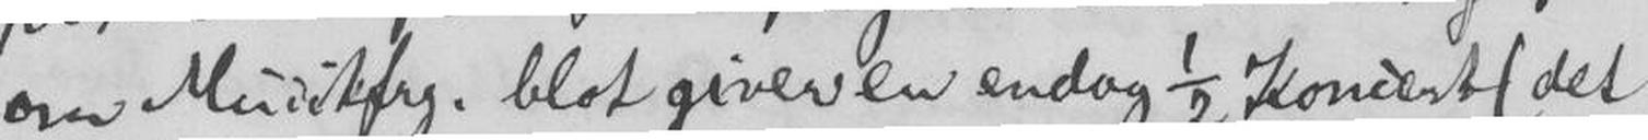om Musikfrg. blot giver en endog 1/2 Konsert (det
